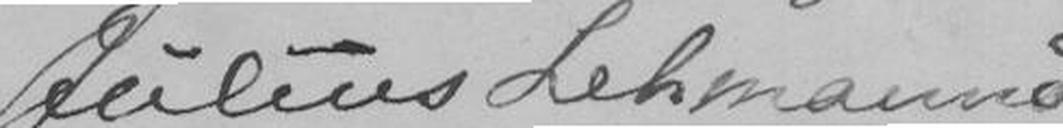Julius Lehmann
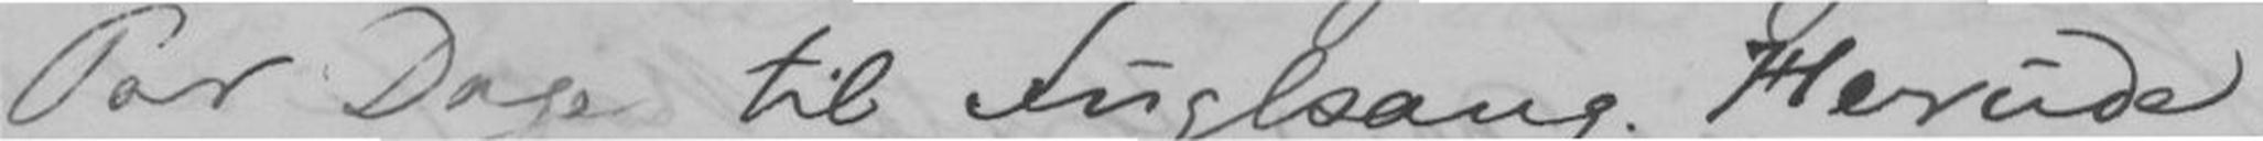Par Dage til Fuglsang. Herude
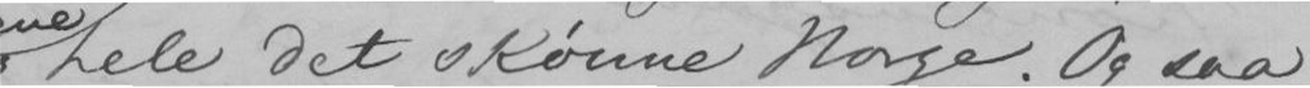hele det skønne Norge. Og saa
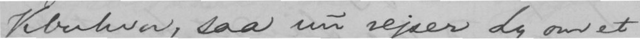Kbnhvn, saa nu rejser Ly om et
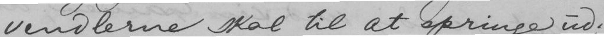vendlerne skal til at springe ud;
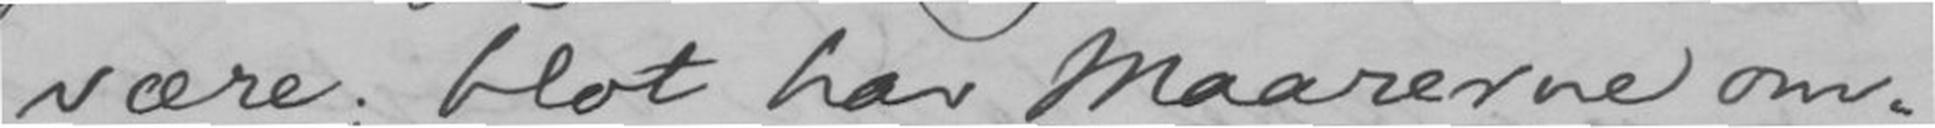være; blot har Maarerne om-
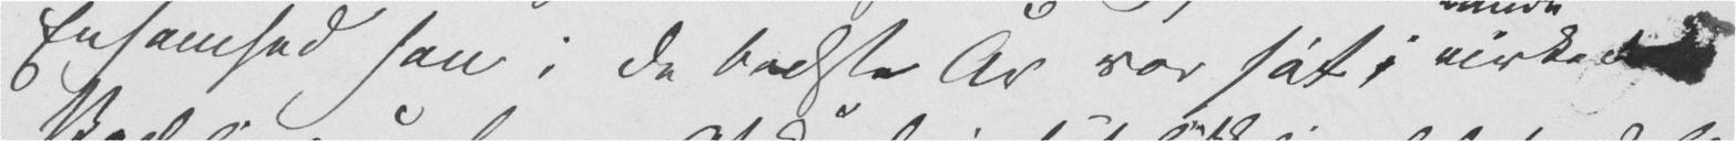Ensomhed han i de bedste År var såt; virke det
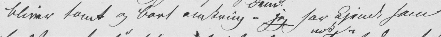bliver tomt og bart omkring – jeg har kjendt ham
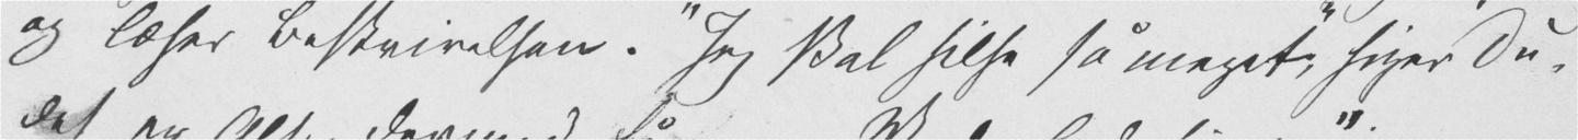og læser Beskrivelsen. "Jeg skal hilse så meget", siger Du,
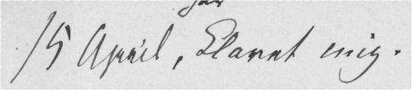15 April, klaret mig.
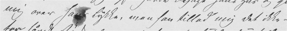mig over hans Lykke, men han tillod mig det ikke –
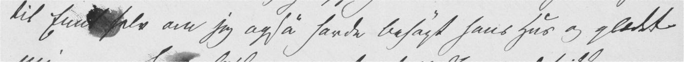til Emil selv om jeg også havde besøgt hans Hus og glædet
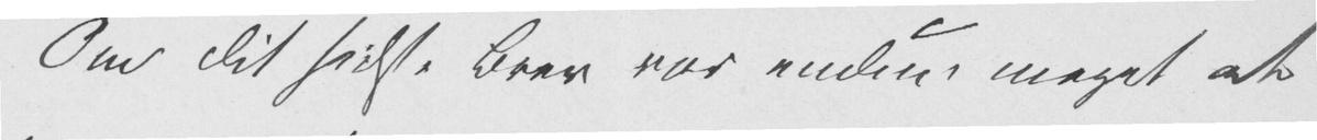Om Dit sidste Brev var endnu meget at
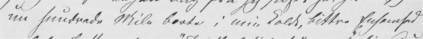nu hundrede Mile borte i min kolde, bittre Ensomhed
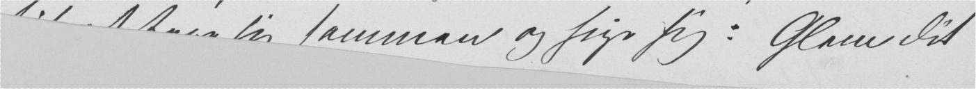til Reae sie sammen og sige sig: Glem Dit
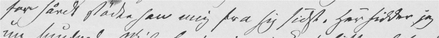for hårdt stødte han mig fra sig sidst. Her sidder jeg
